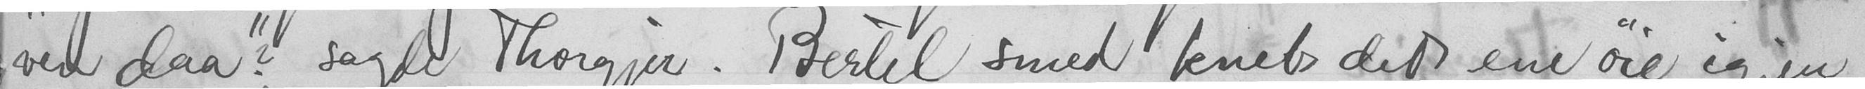ven daa?" sagde Thorgjer. Bertel smed kneb det ene øie igjen
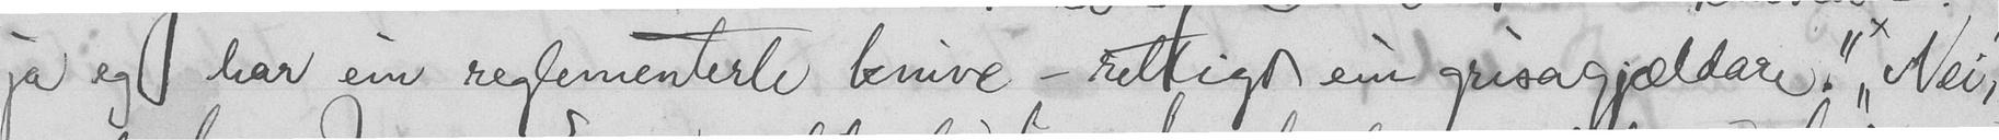ja eg har ein reglementerte knive - rettigt ein grisagjældare! "Nei",
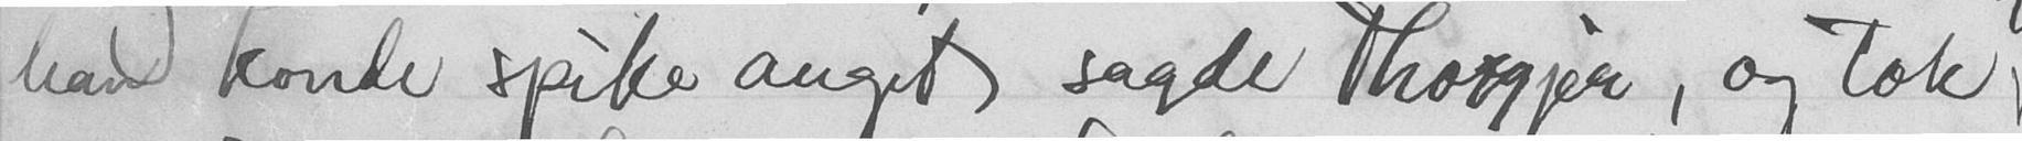han konde spike auget sagde Thorgjer, og tok
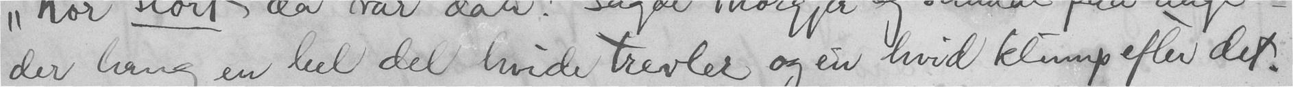der hang en hel del hvide trevler og ein hvid klump efter det.
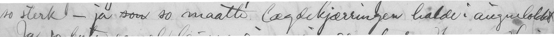jo sterk - ja  so maatte lægdekjærrigen halde i augnelokket
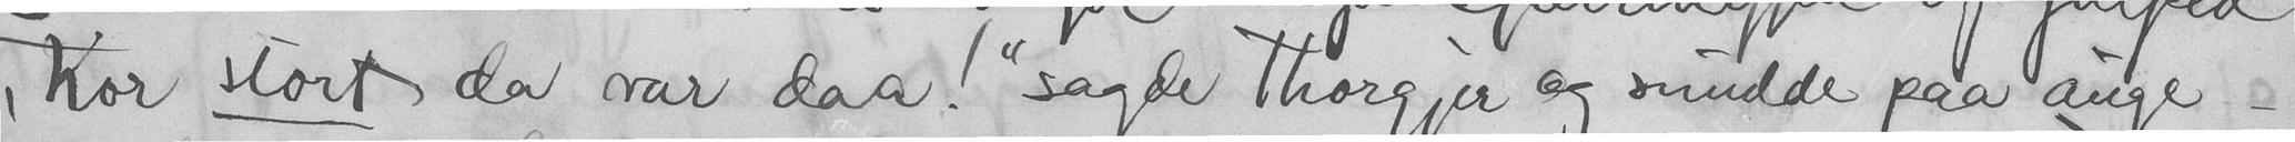"Kor stort da var daa!" sagde Thorgjer og snudde paa auge -
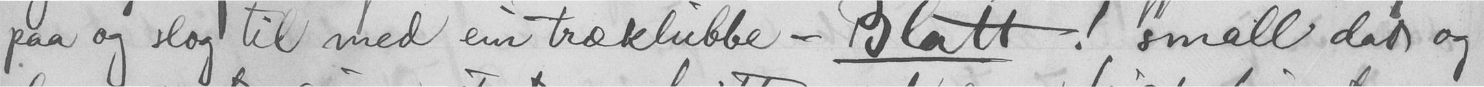paa og slog til med ein træklubbe - Blatt! small dat og
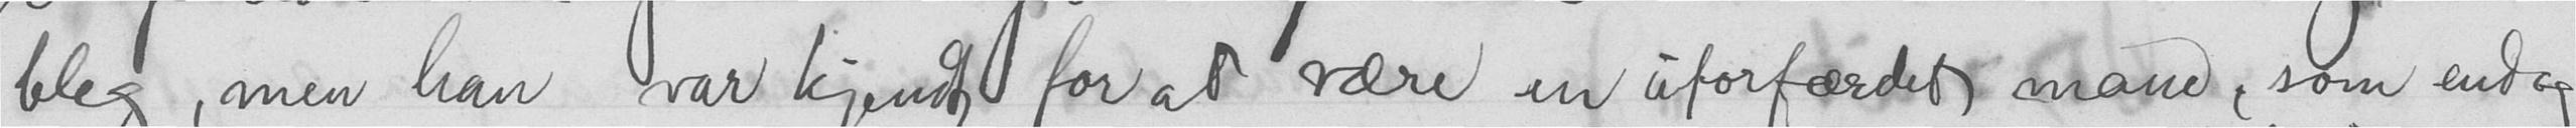bleg, men han var kjendt forat være en uforfærdet mann, som endog
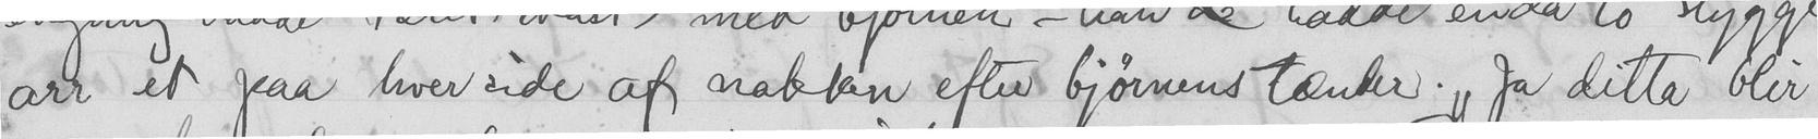arr et paa hver side af nakken efter bjørnens tænder. "Ja dette blir
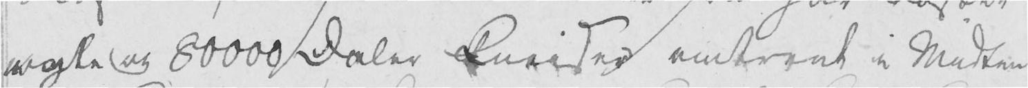nogle og 80000 Daler kneiser omtrent i Midten
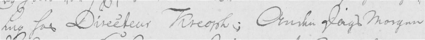lyp hos Directeur Kreoph; Anden Dags Morgen
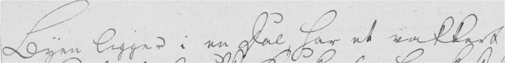Byen ligger i en Dal, har et vakkert
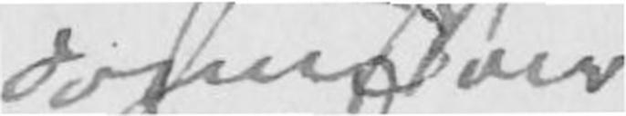oversitter
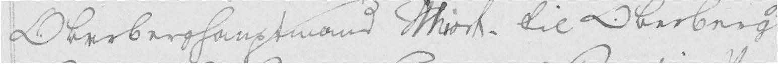Oberberghauptmand Hiort, til Oberberg
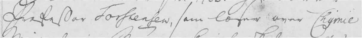Professor Torstensen, som læser over Chymie
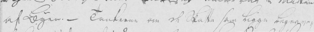af Byen. - Tankerne om de Skatte som ligge begrave-
